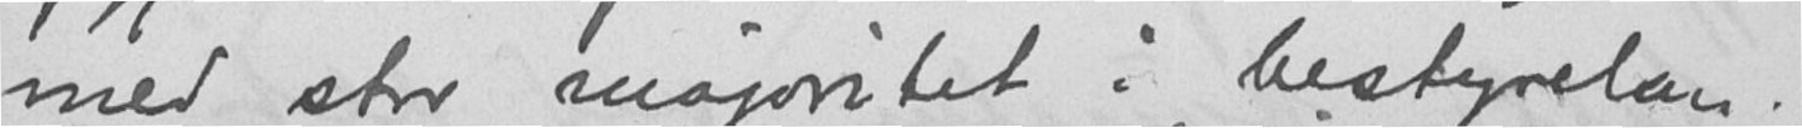med stor majoritet i bestyrelsen.
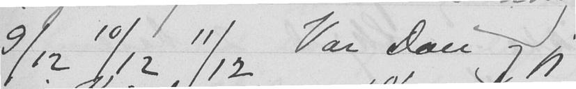9/12 10/12 11/12 Var Don og jeg
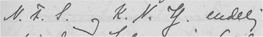N. F. S. og K. N. Y. endelig
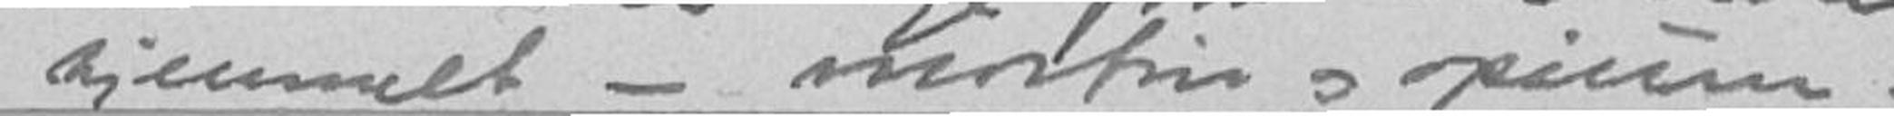hjemmet - morfin og opium.
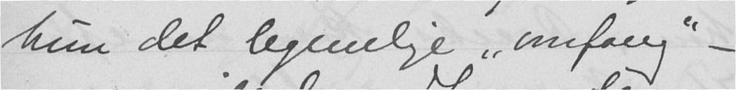hun det legemlige "omfang" -
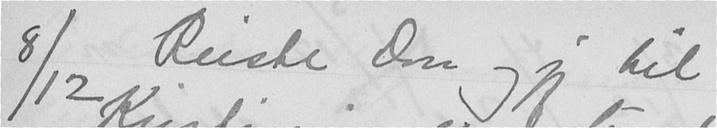8/12 Reiste Don og jeg til
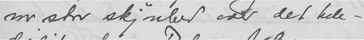var stor skjønhed over det hele -
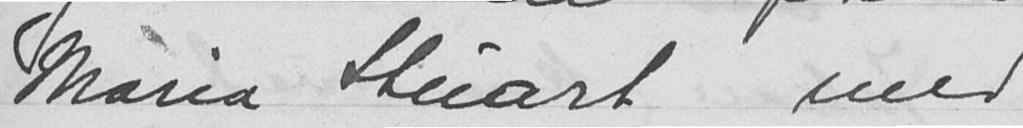Maria Stuart med
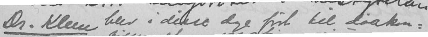Dr. Klem blev i disse dage ført til diakon-
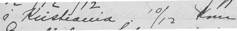i Kristiania. 10/12 Kom
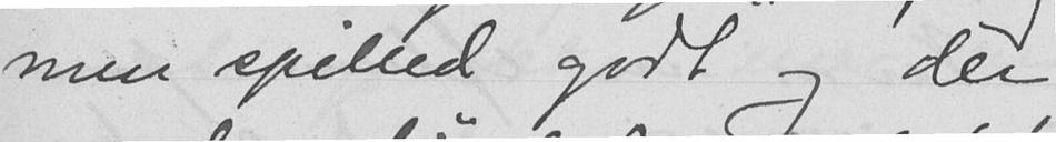men spilled godt og der
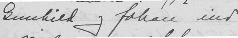Gunhild og Johan ind
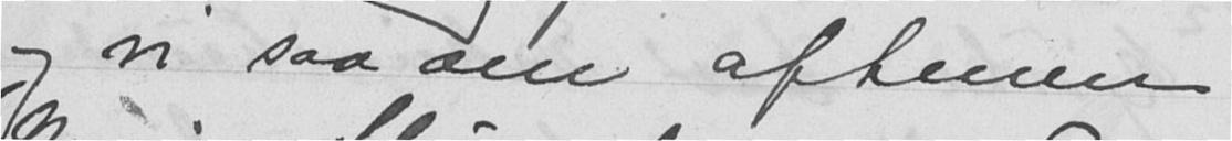og vi saa om aftenen
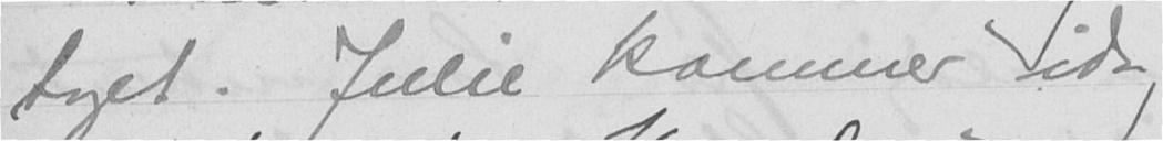toget. Julie kommer idag.
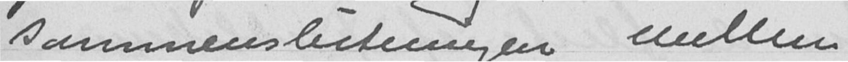sammenslutningen mellem
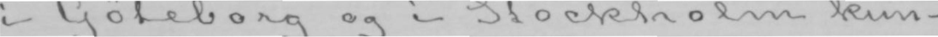i Göteborg og i Stockholm kun-
